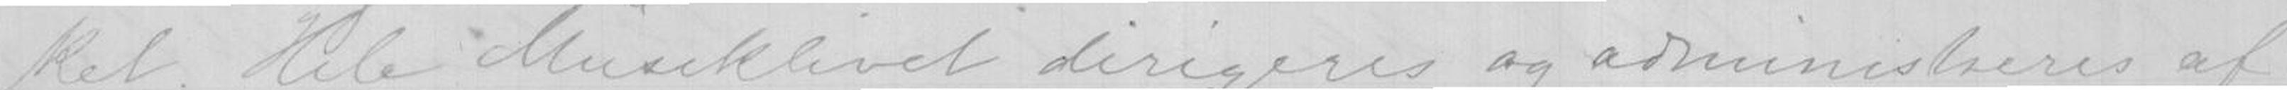ket. Hele Musiklivet dirigeres og administreres af
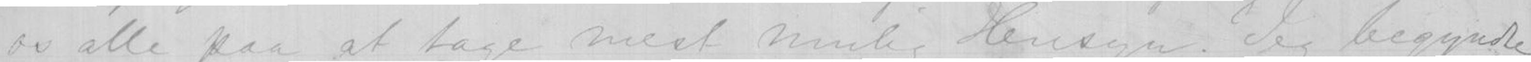os alle paa at tage mest mulig Hensyn. Jeg begyndte
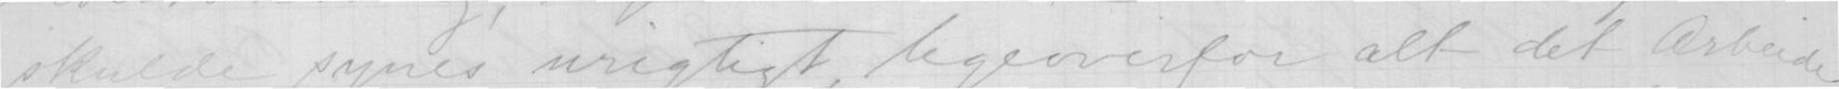skulde synes urigtigt, ligeoverfor alt det Arbeide
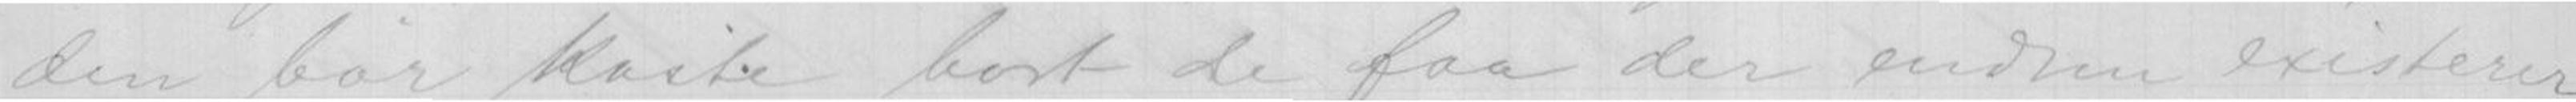den bør kaste bort de faa der endnu existerer
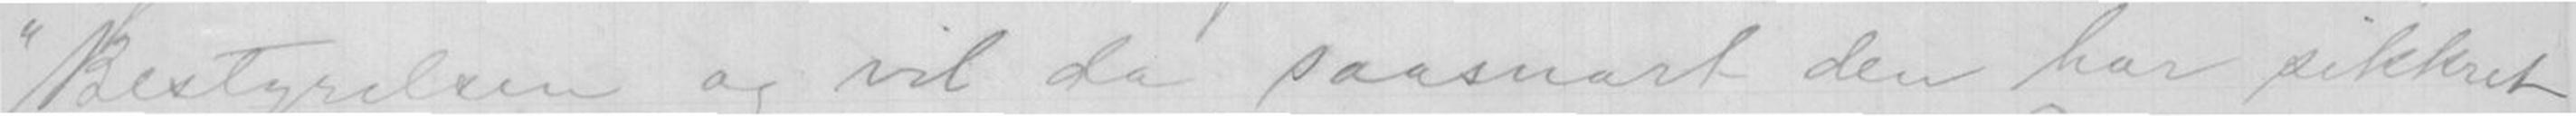Bestyrelsen og vil da, saasnart den har sikkret
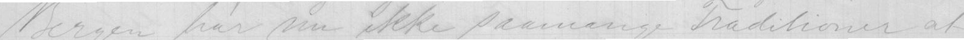Bergen har nu ikke saamange Traditioner, at
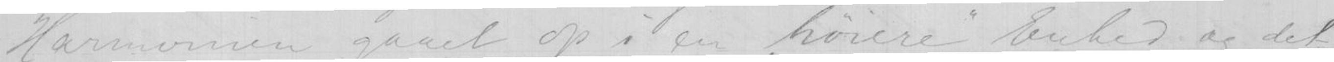Harmonien gaaet op i en høiere Enhed og det
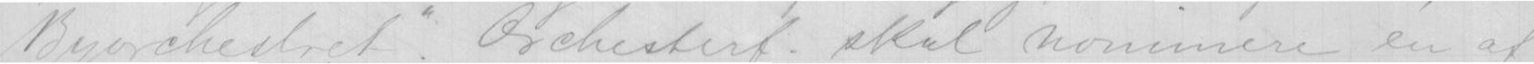Byorchestret. Orchesterf. skal nominere én af
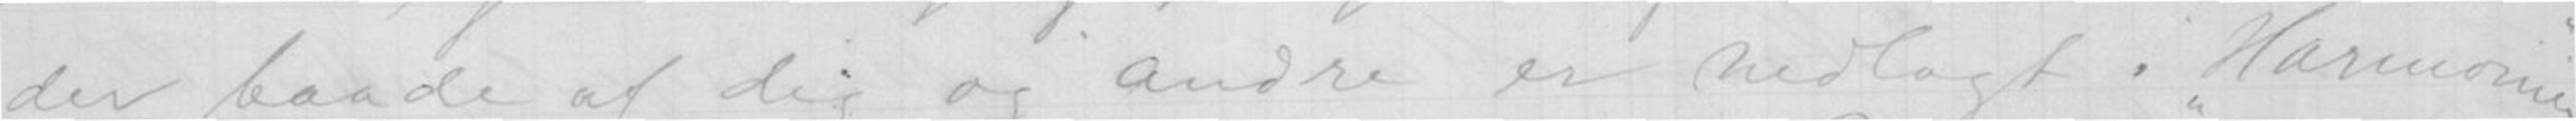der baade af dig og andre er nedlagt i Harmonien
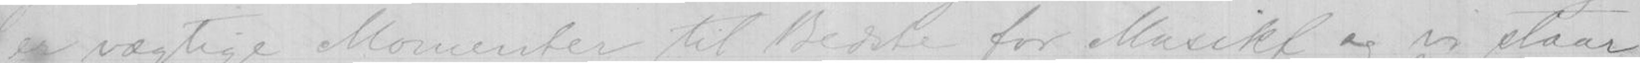er vægtige Momenter til Bedste for Musikf. og vi staar
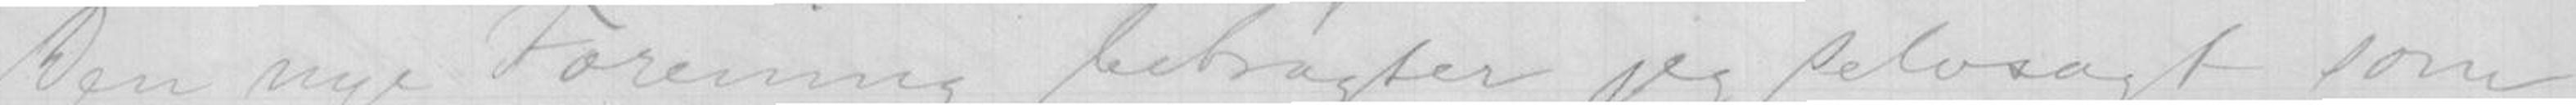Den nye Forening betragter jeg selvsagt som
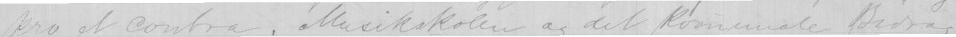pro et Contra. Musikskolen og det komunale Bidrag
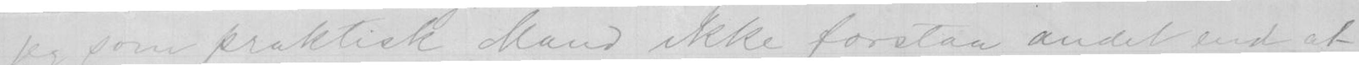jeg som praktisk Mand ikke forstaa andet, end at
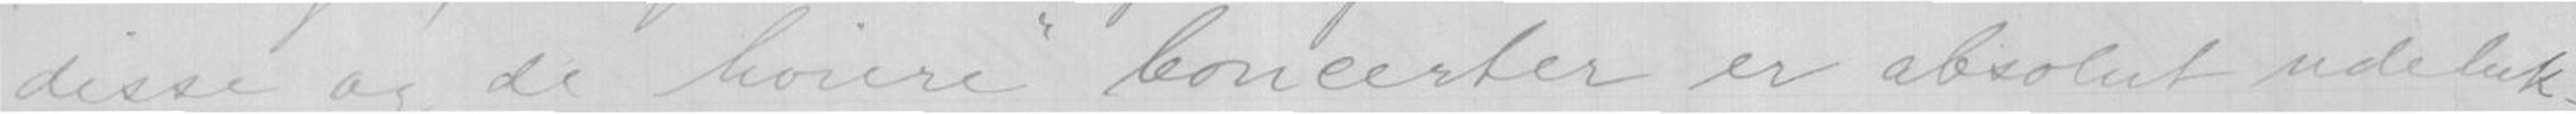disse og de høiere Concerter er absolut udeluk-
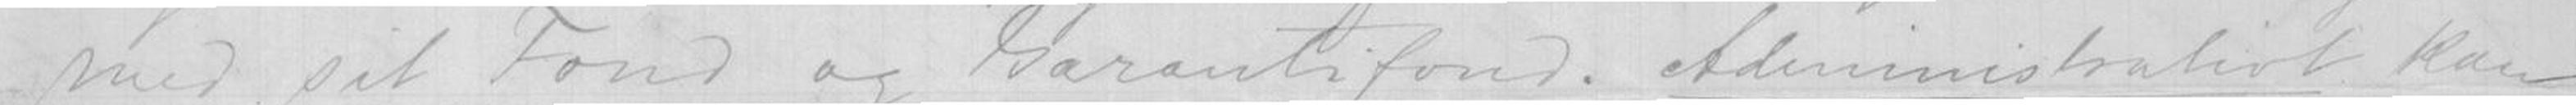med sit Fond og Garantifond. Administrativt kan
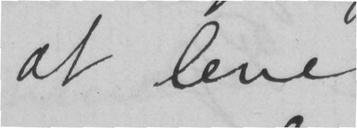at leve
